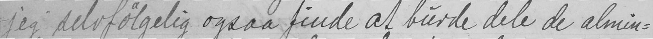jeg selvfølgelig ogsaa finde at burde dele de almin-
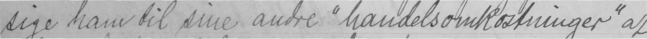sige ham til sine andre "handelsomkostninger" at
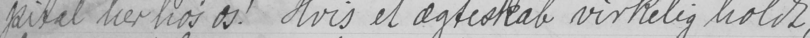pital her hos os! Hvis et ægteskab virkelig holdt,
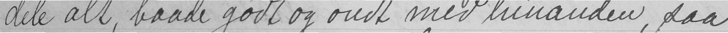dele alt, baade godt og ondt med hinanden, saa
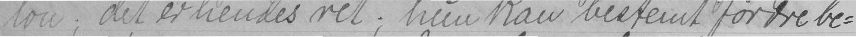løn; det er hendes ret; hun kan bestemt førdre be-
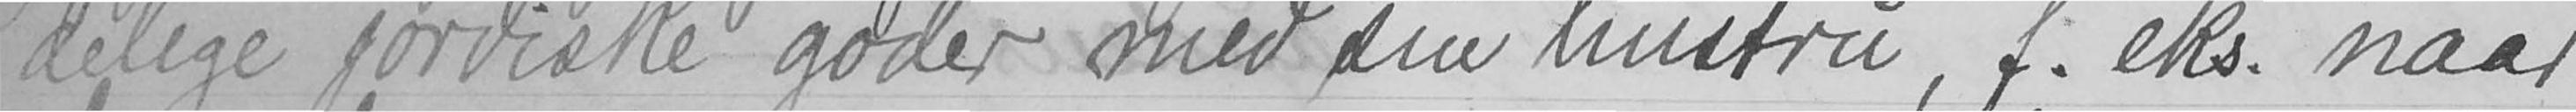delige jordiske goder med sin hustru, f. eks. naar
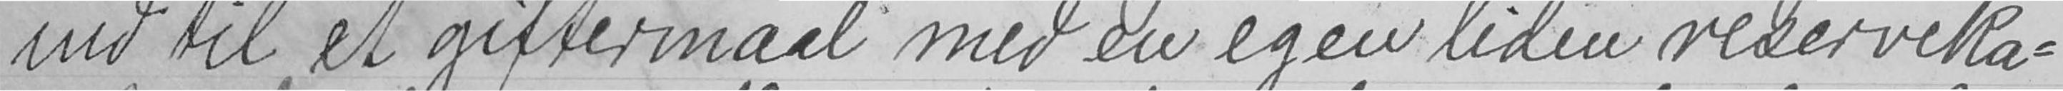ind til et giftermaal med en egen liden reserveka-
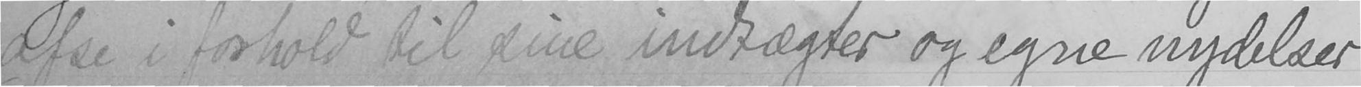afse i forhold til sine indtægter og egne nydelser
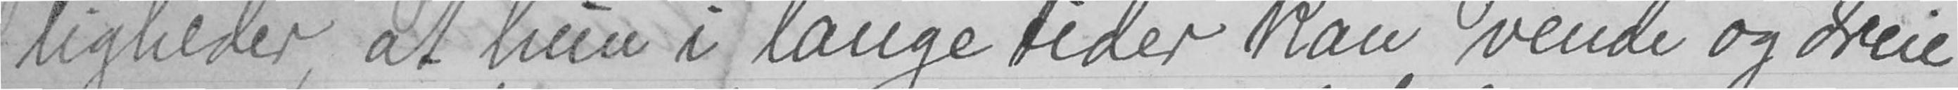ligheder, at hun i lange tider kan vende og dreie
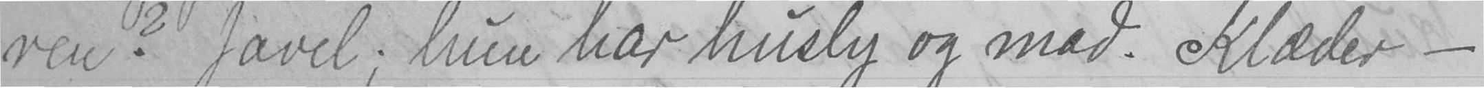ren? Javel; hun har husly og mad. Klæder -
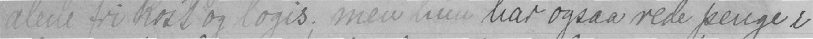alene fri kost og logis; men nu har ogsaa rede penge i
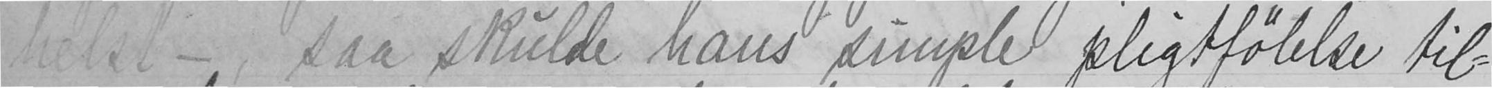helst -, saa skulde hans simple pligtfølelse til-
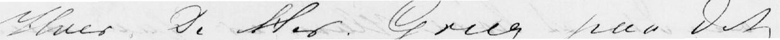Hvor De Her Grieg paa det
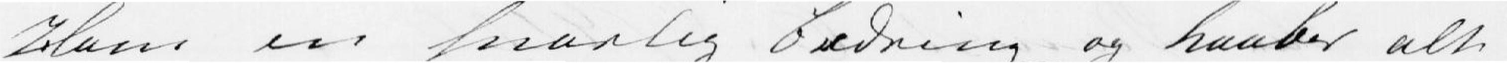ham en snarlig bedring og haaber alt
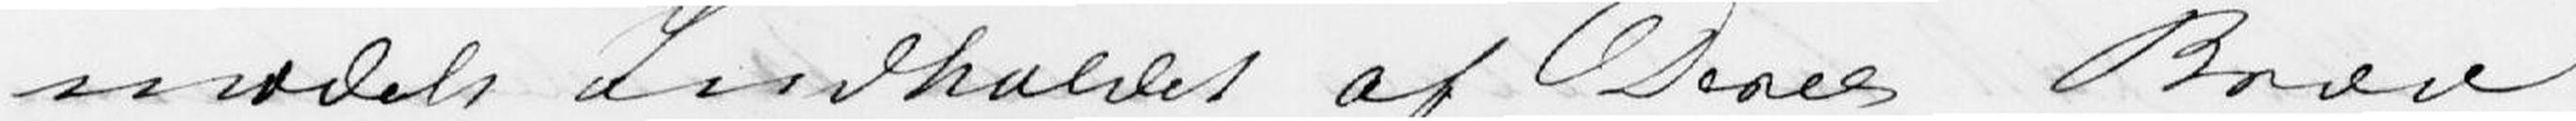medelt Indholdet af Deres Breve
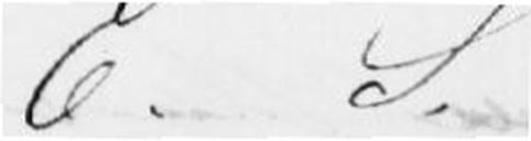E. S.
